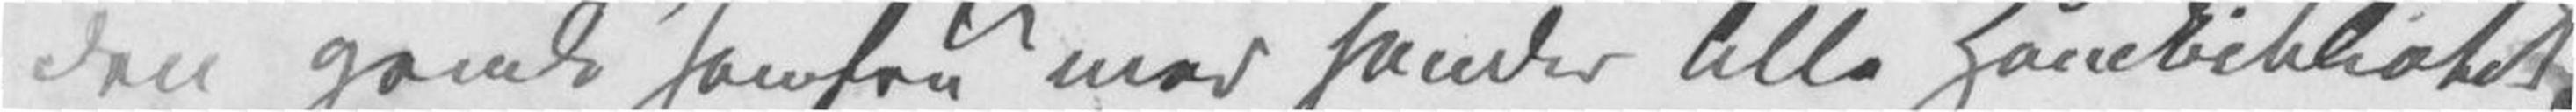den gamle Jomfru med hendes lille Håndbibliotek.
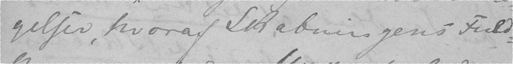gelser, hvoraf Skabningens Fuld-
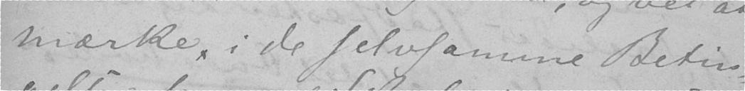mærke, i de selvsamme Betin-
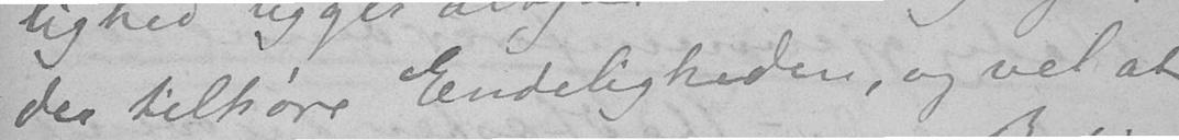der tilhøre Endeligheden, og vel at
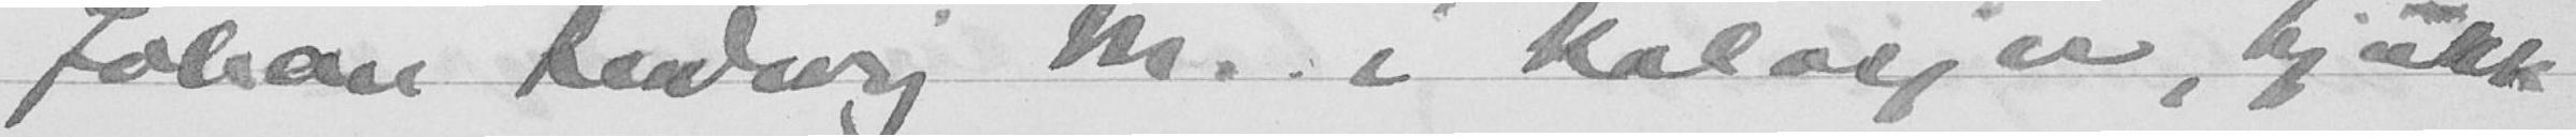Johan Hedvig M. i kolasjer, kjøkk
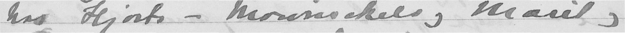har Hjorts - Mowinckels og Marit og
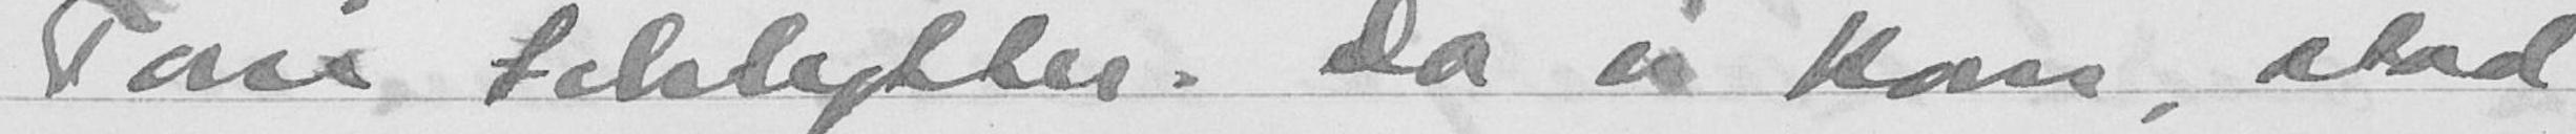Tore Fehlyttes. Da vi kom, stod
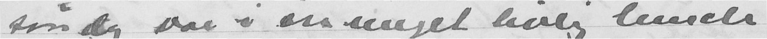søndag var i sin meget livlig lemcls
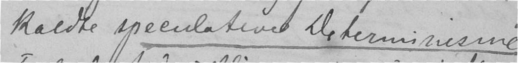kaldte speculative Determinisme
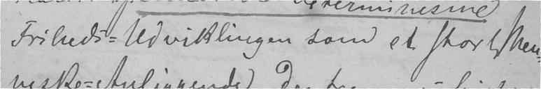Friheds-Udviklingen som et stort Men-
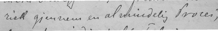risk gjennem en almindelig Proces,
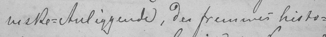neske-Anliggende, der fremmes histo-
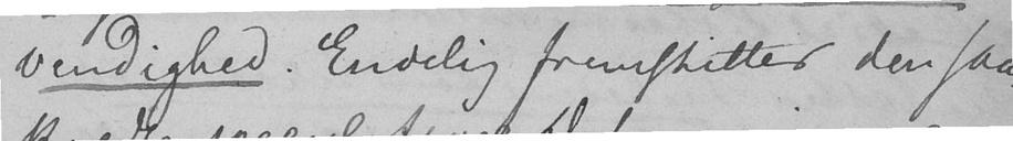vendighed. Endelig fremstiller den saa-
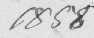1858
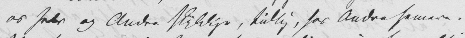os selv og Andre skyldige, tidlig, hos Andre senere.
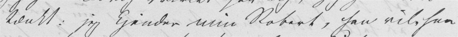tænkt: jeg kjender min Robert, han vil, han
